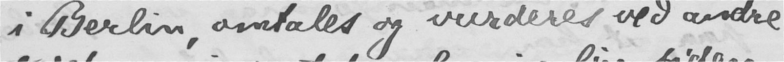i Berlin, omtales og vurderes ved andre
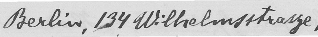Berlin, 134 Wilhelmsstrasse,
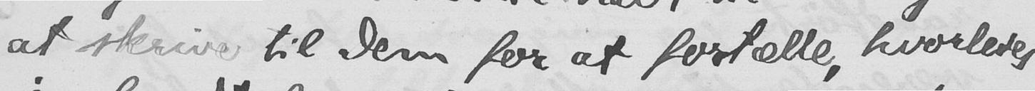at skrive til Dem for at fortælle, hvorledes
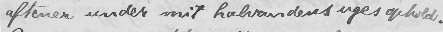aftener under mit halvandens uges ophold.
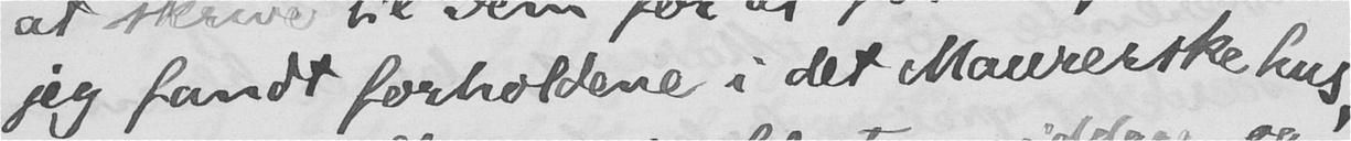jeg fandt forholdene i det Maurerske hus,
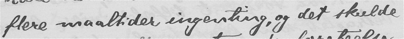flere maaltider ingenting, og det skulde
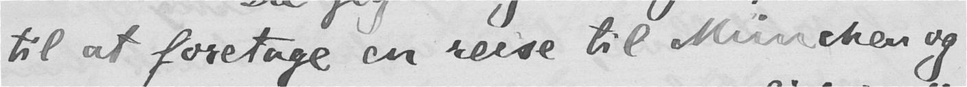til at foretage en reise til München og
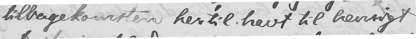tilbagekomsten hertil havt til hensigt
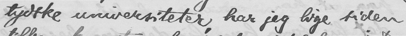tydske universiteter, har jeg lige siden
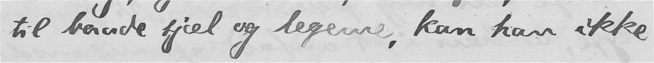til baade sjæl og legeme, kan han ikke
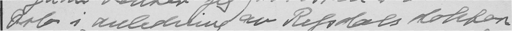Oslo i anledning av Refsdals doktor-
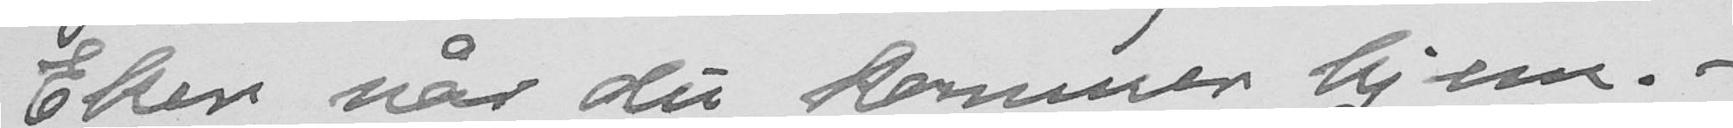Eker når du kommer hjem.-
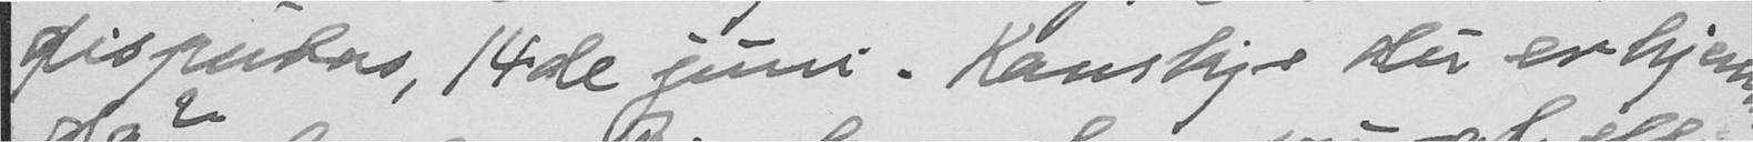disputas, 14de juni. Kanskje du er hjemme
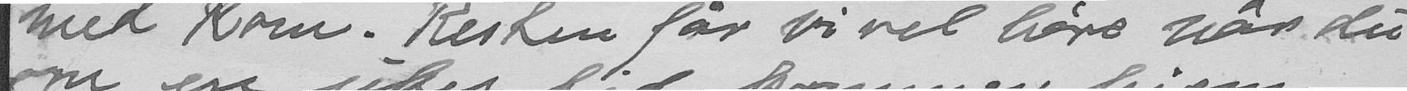med Rom. Nesten får vi vel høre når du
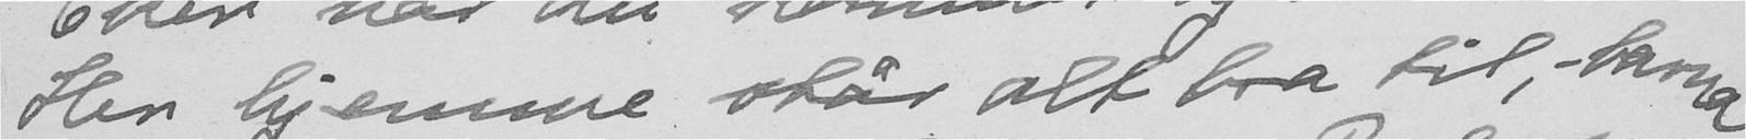Her hjemme står alt bra til, - barna
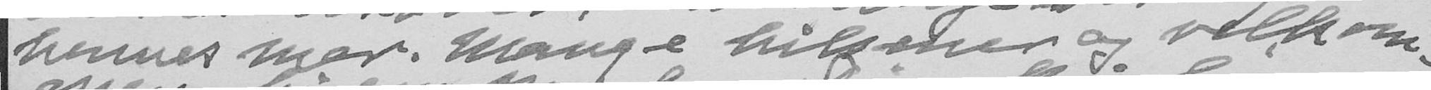hennes mor. Mange hilsener og velkom-
